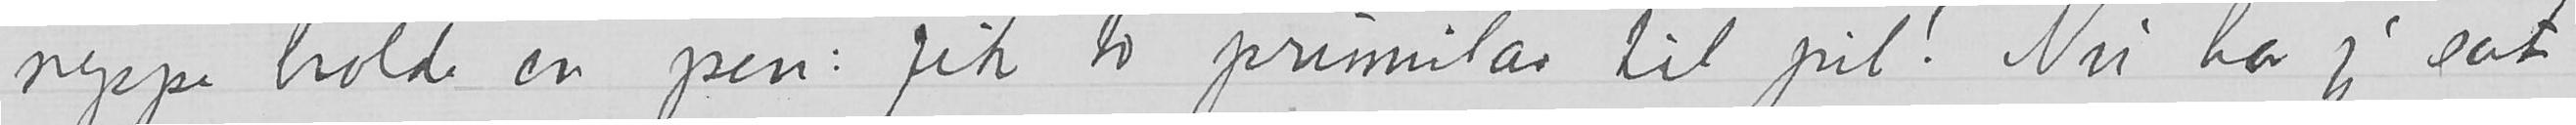neppe holde en pen: fik to primular til jul! Nu har jeg sat
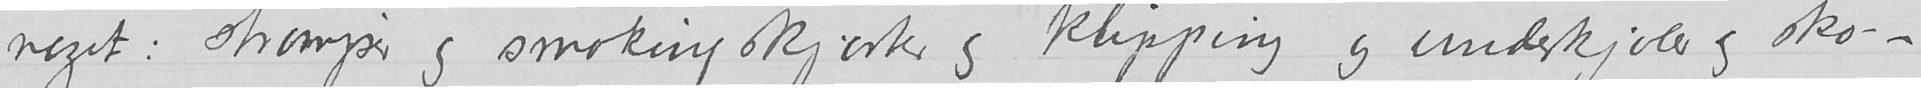noget: strømper og smokingskjorter og klipping og underkjoler og sko –
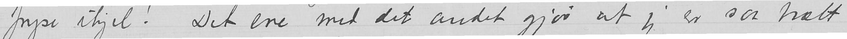fryse i hjel! Det ene med det andet gjør at jeg er saa trætt
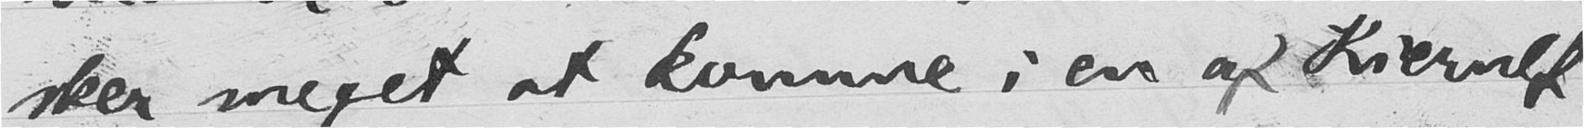sker meget at komme i en af Kierulf
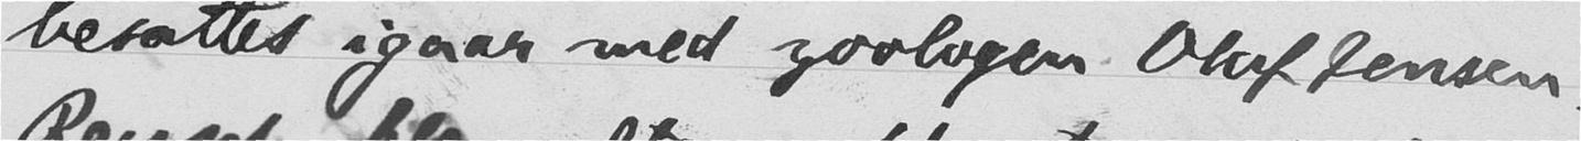besattes igaar med zoologen Olaf Jensen.
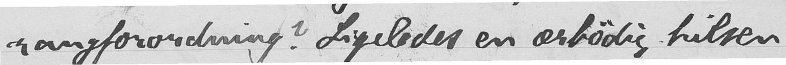rangforordning? Ligeledes en ærbødig hilsen
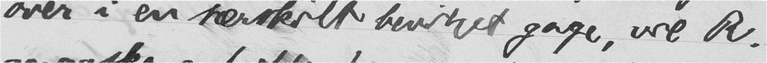over i en særskilt bevirket gage, vil R.
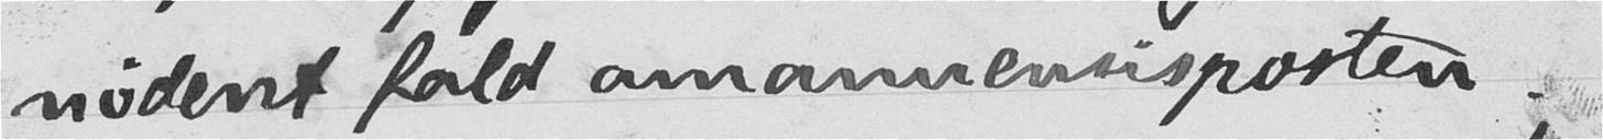nødent fald amanuensisposten.
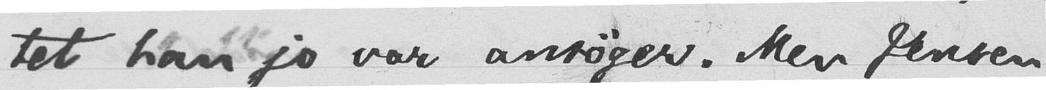tet han jo var ansøger. Men Jensen
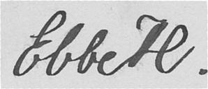Ebbe H.
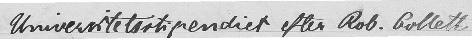Universitetsstipendiet efter Rob. Collett
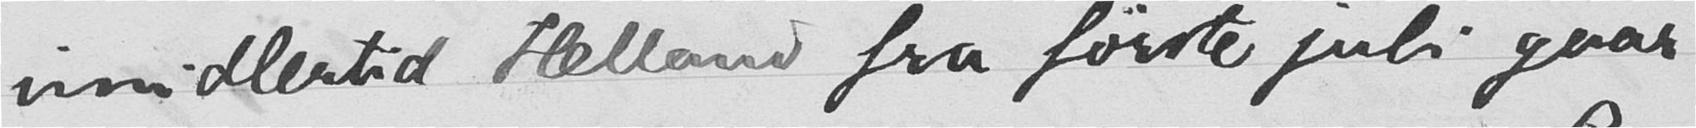imidlertid Helland fra første juli gaar
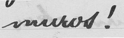muros!
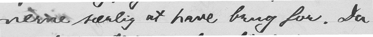nerne særlig at have brug for. Da
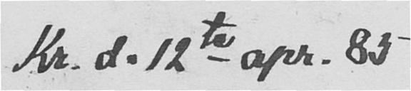Kr. d. 12te apr. 85.
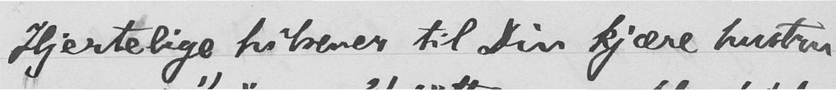Hjertelige hilsener til Din kjære hustru
